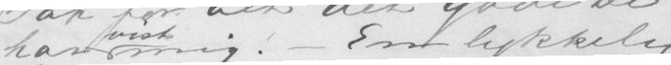har mig.- En lykkelig
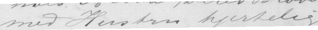med Hustru hjertelig
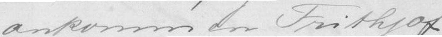ankommen Frithjof
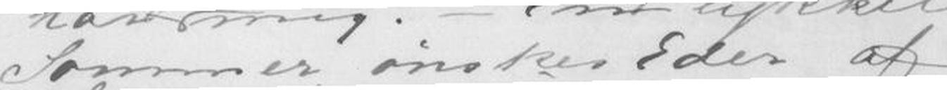Sommer ønskes Eder af
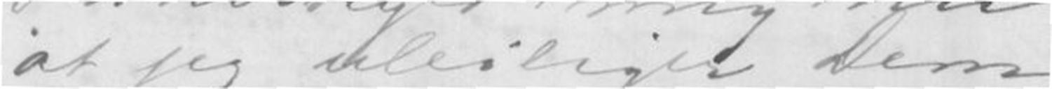at jeg uleiliger Dem,
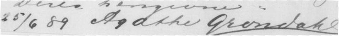25/6 89 Agathe Grøndahl
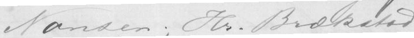Nansen; Hr. Brækstad
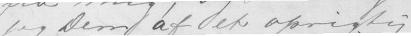jeg Dem af et oprigtig
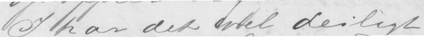I har det vel deiligt
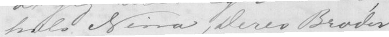hils Nina, Deres Broder
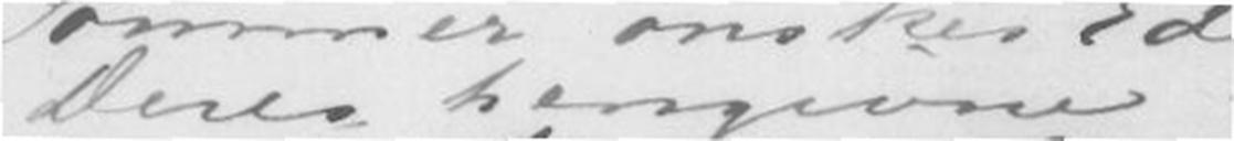Deres hengivne
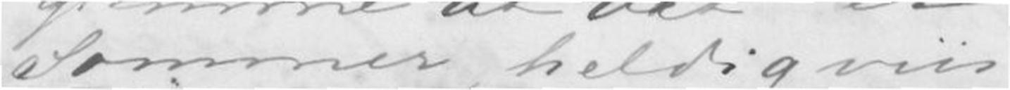Sommer, heldigviis
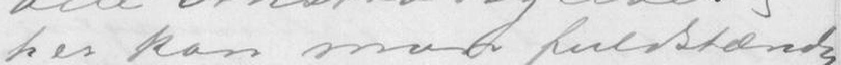her kan man fullstændig
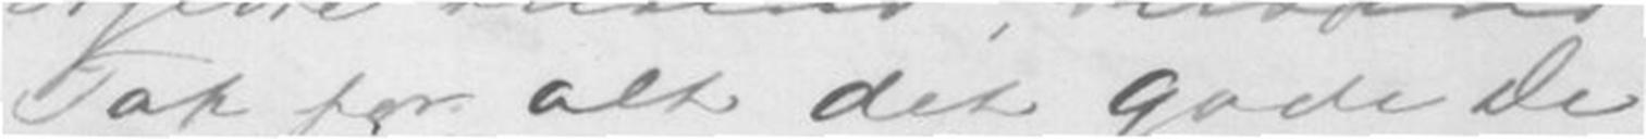Tak for alt det Gode De
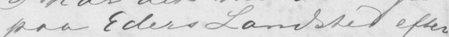paa Eders Landsted efter
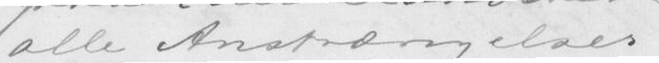alle Anstrængelser,
